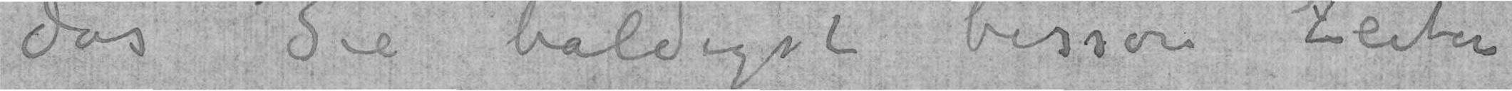das Sie baldigst bessere Zeiten
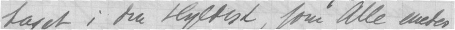taget i den Hyldest som Alle enedes
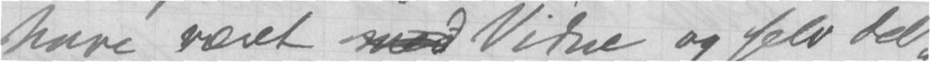have været Vidne og selv del-
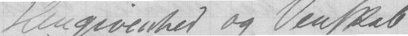I Hengivenhed og Venskab
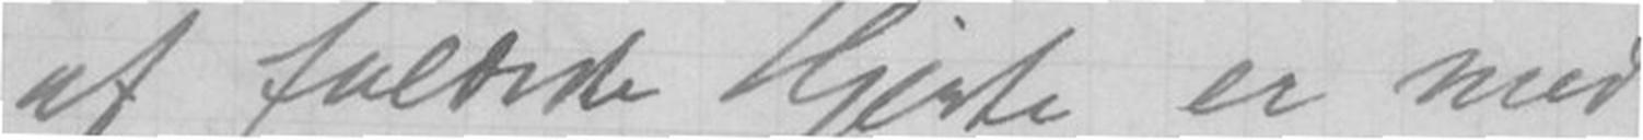af fuldeste Hjerte er med i
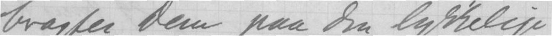bragtes Dem paa den lykkelige
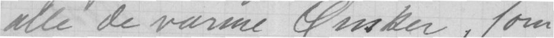alle de varme Ønsker, som
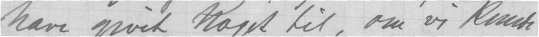have givet Noget til, om vi kunde
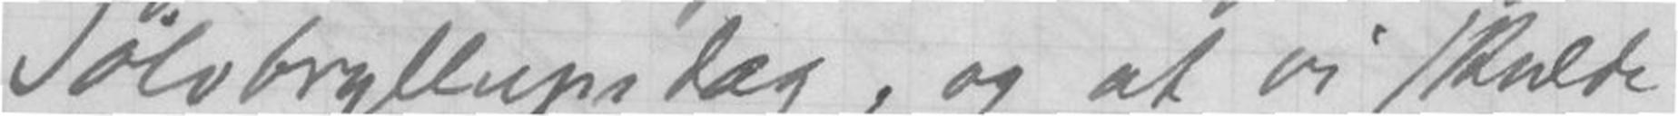Sølvbryllupsdag, og at vi skulde
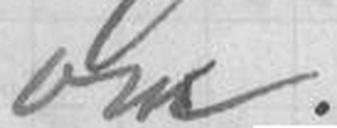om.
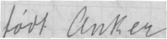født Anker
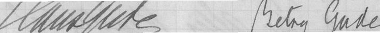Hans GudeBetsy Gude
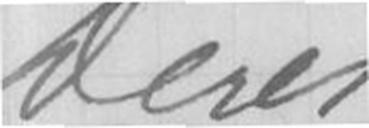Deres
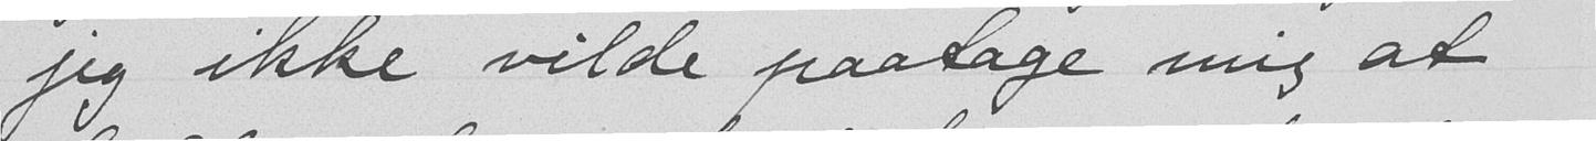jeg ikke vilde paatage mig at
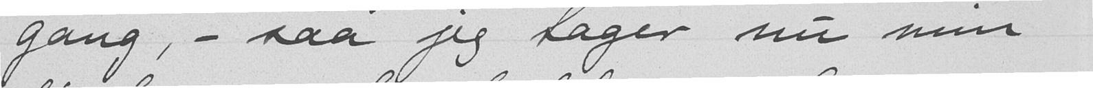gang, - saa jeg tager nu min
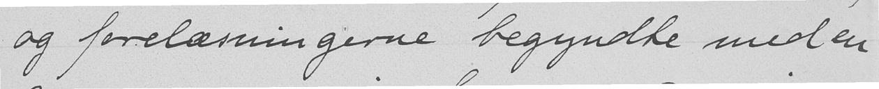og forelæsningerne begyndte med en
